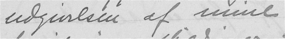udgivelsen af mine
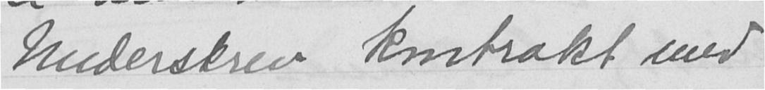Underskrev kontrakt med
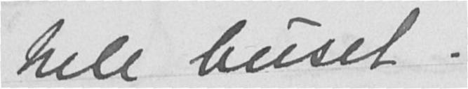hele huset.
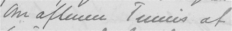Om aftenen Tennis af
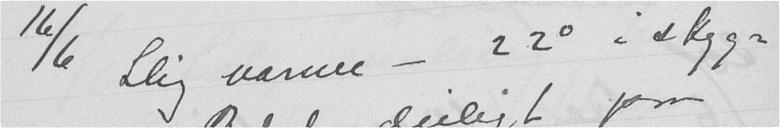16/6 Slig varme - 22° i skyg-
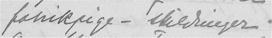fabrikpige-skildringer.
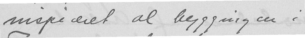inspiceret al byggingen i
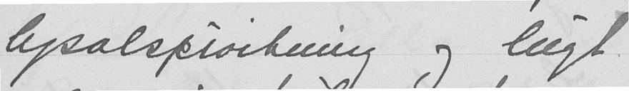lysolsprøitning og lugt
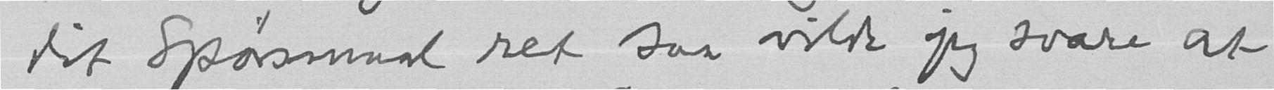dit Spørsmaal ret saa vilde jeg svare at
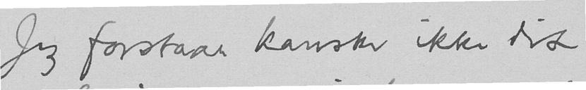Jeg forstaar kanske ikke dit
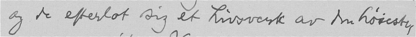og de efterlot sig et Livsverk av den høieste,
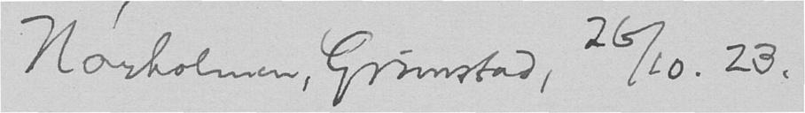Nørholm, Grimstad, 26/10. 23.
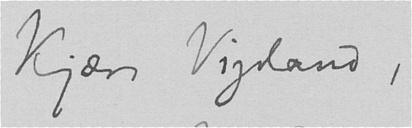Kjære Vigeland,
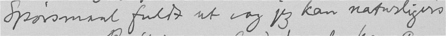Spørsmaal fuldt ut og jeg kan naturligvis
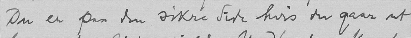Du er paa den sikre Side hvis du gaar ut
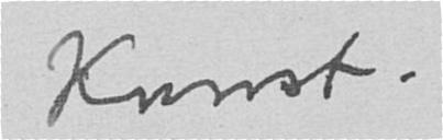Kunst.
